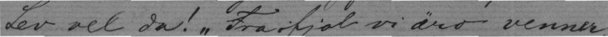Lev vel da! Fra ifjol vi äro venner,
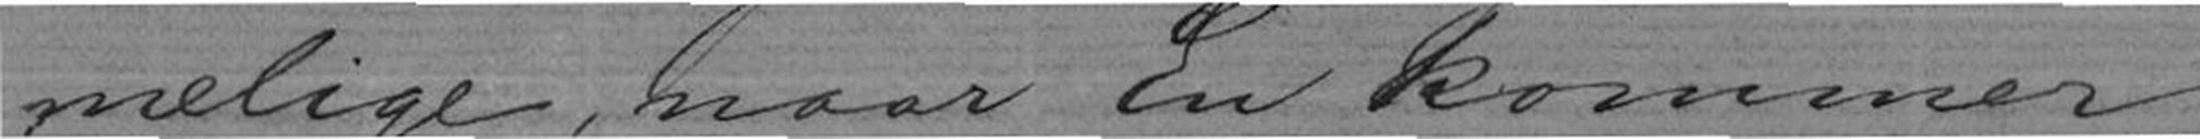melige, naar En kommer
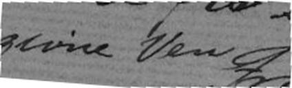givne Ven
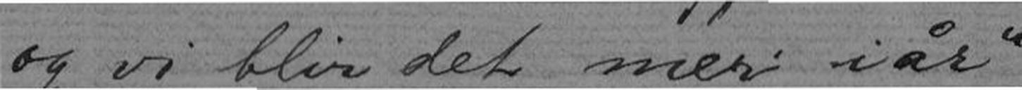og vi blir det mer iår.
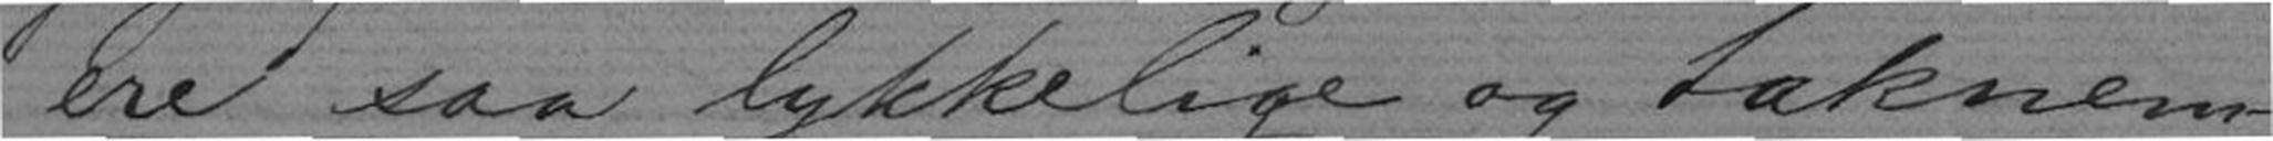ere saa lykkelige og taknem-
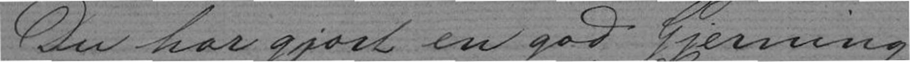Du har gjort en god Gjerning
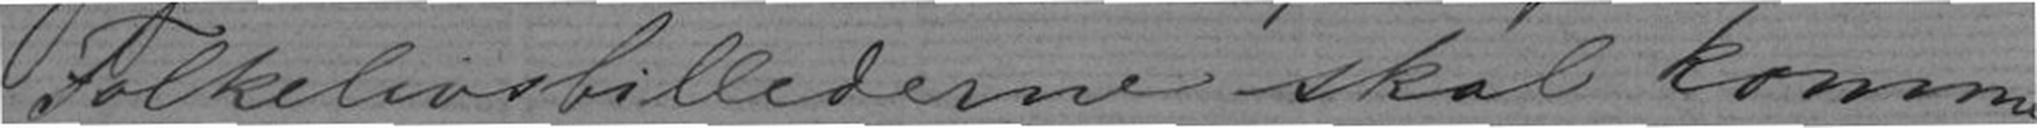Folkelivsbillederne skal komme
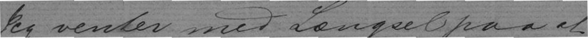Jeg venter med Længsel paa at
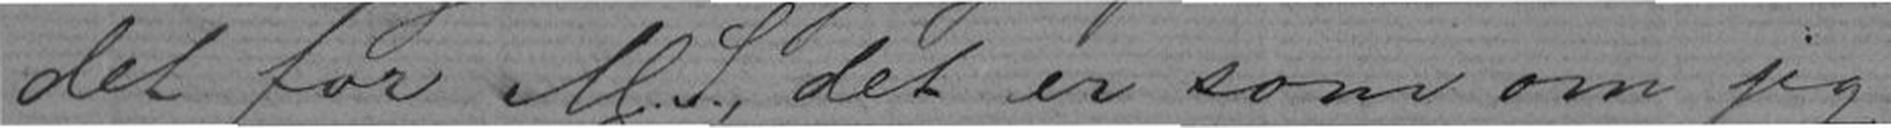det for M.S., det er som om jeg
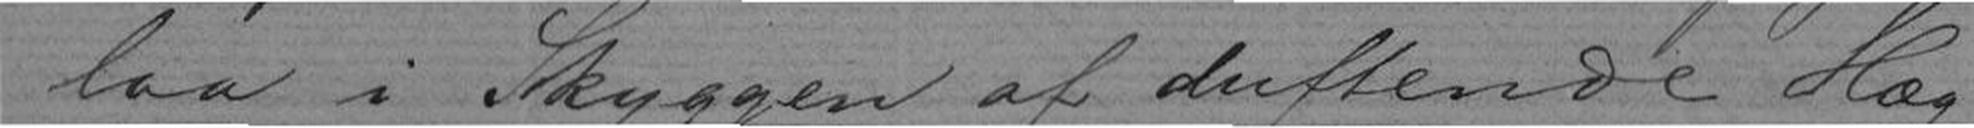laa i Skuggen af duftende Hæg
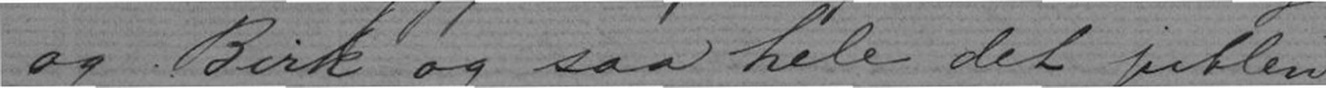og Birk og saa hele det jublen-
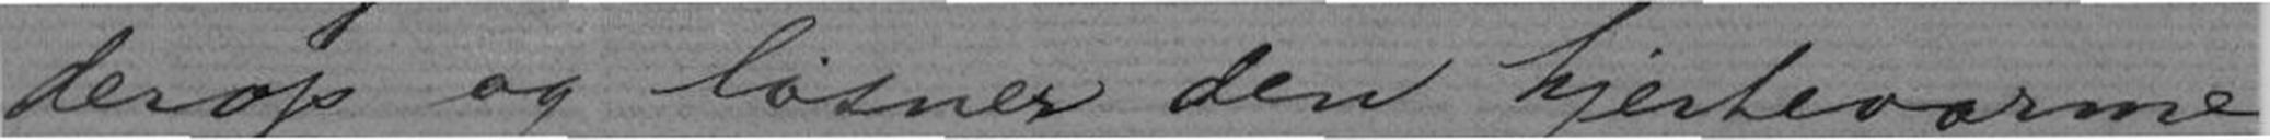derop og løsner den hjertevarme
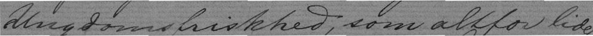Ungdomsfriskhed, som altfor lidet
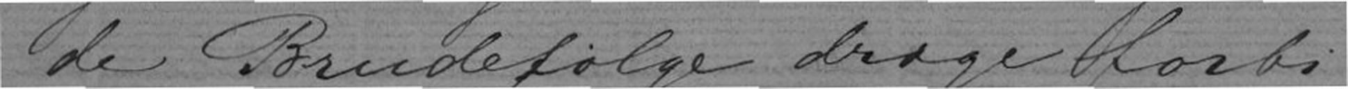de Brudefølget drage forbi
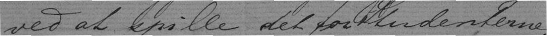ved at spille det for Studenterne;
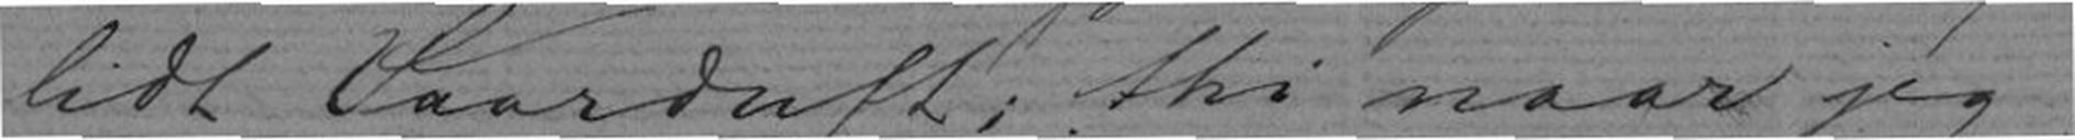lidt Vaarduft; thi naar jeg
